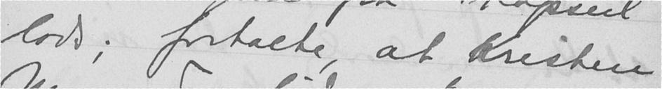lads; fortalte, at Kristen
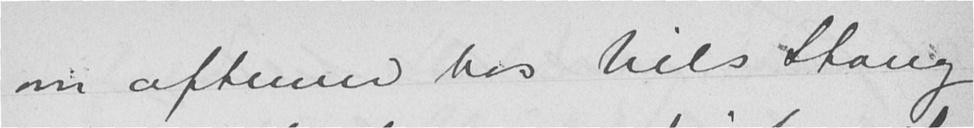om aftenen hos Nils Stang
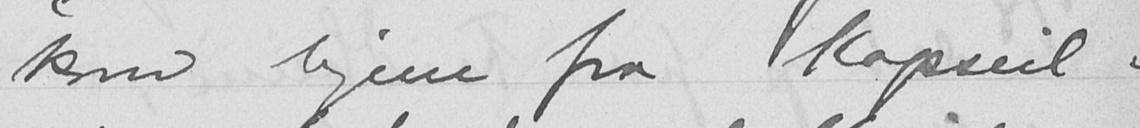kom hjem fra kapseil-
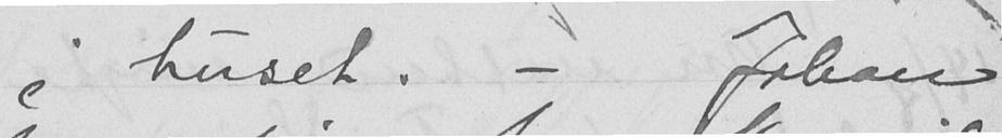i huset. - Johan
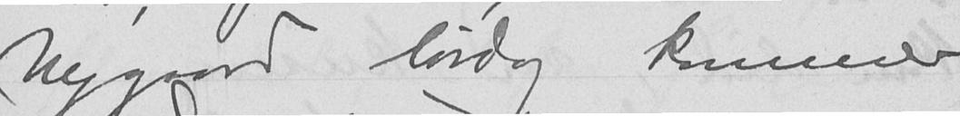Nygaard lørdag kommer
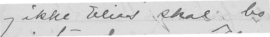og ikke Elias skal bo
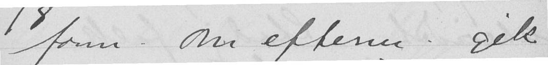form. Om efterm. gik
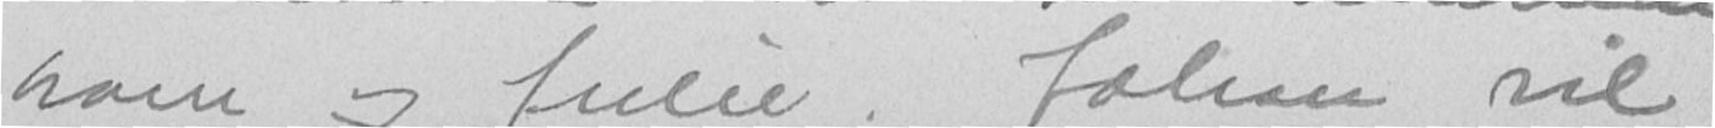ham og Julie. Johan vil
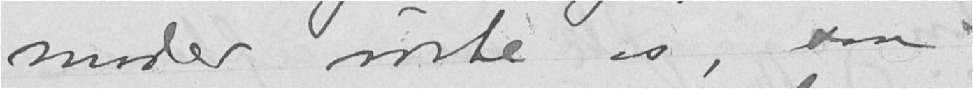moder rørte os, saa
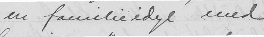en familieidyl med
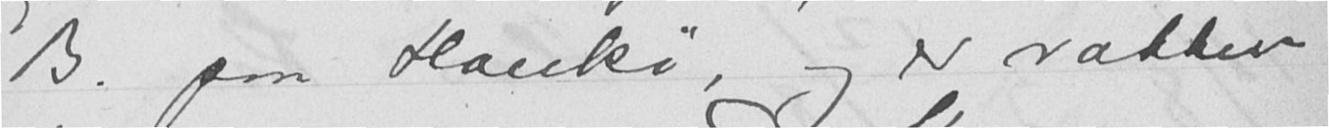B. paa Hankø, og er ratten
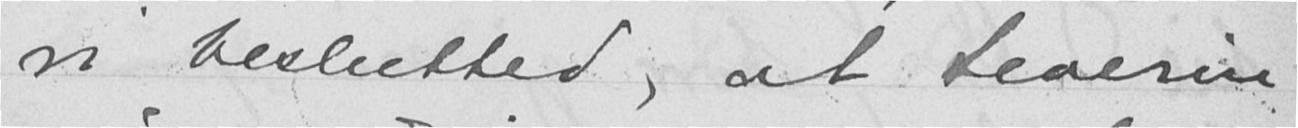vi beslutted, at Severin
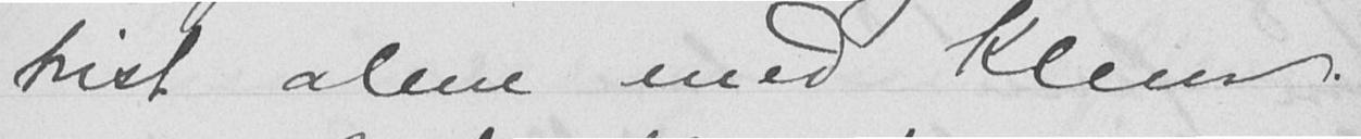trist alene med Klem.
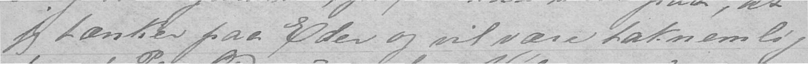jeg tænker paa Eder og vil være taknemlig
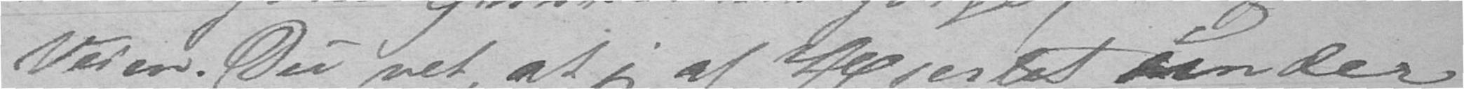Veien. Du vet at jeg af Hjertet under
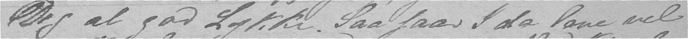Dig al god Lykke. Saa faar I da leve vel
